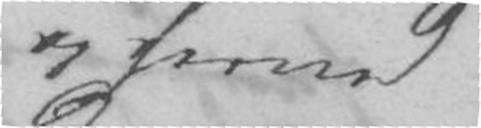og herved
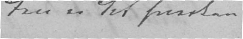Den er det hverken
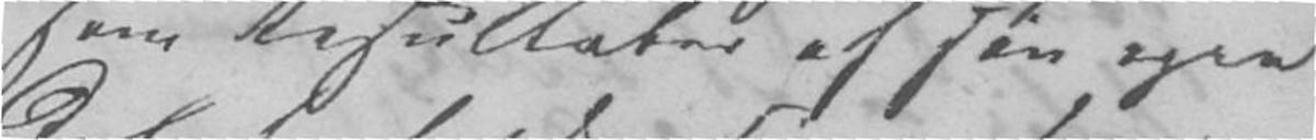som Resultater af sin egen
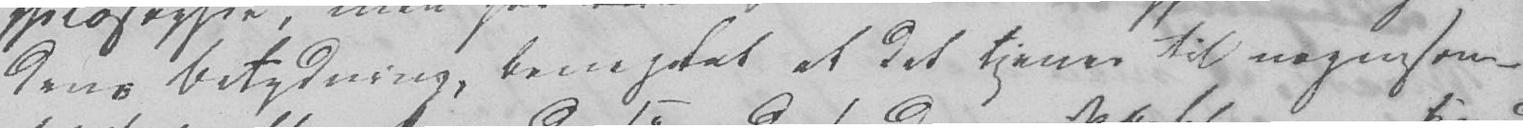dens Betydning, benægtet at det tjener til nogensom-
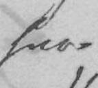hvor
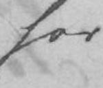har
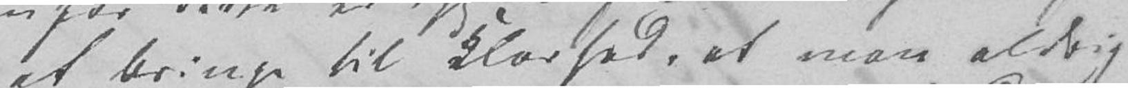at bringe til Klarhed, at man aldrig
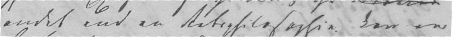andet end en Retsphilosophie kan en
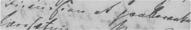Discussion at paaberaabe
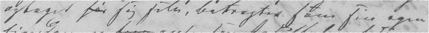optaget for sig selv, betragter som sin egen
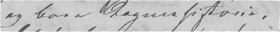og bare Sagnshistorie,
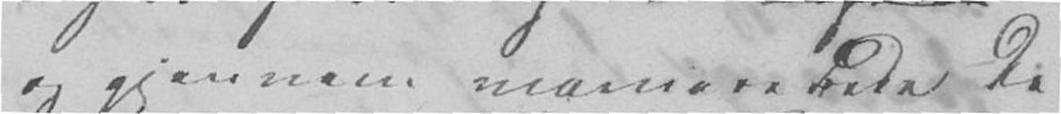og gjennem nærmere De-
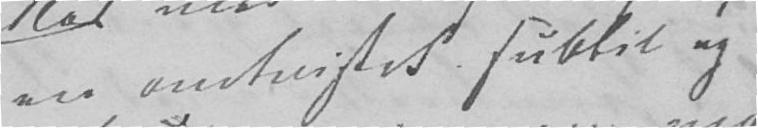en omtvistet subtil og
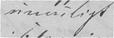umuligt
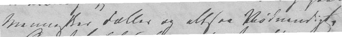Mennesker fælles og altsaa Nødvendigt,
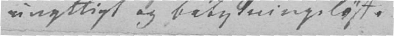unyttigt og betydningsløst.
